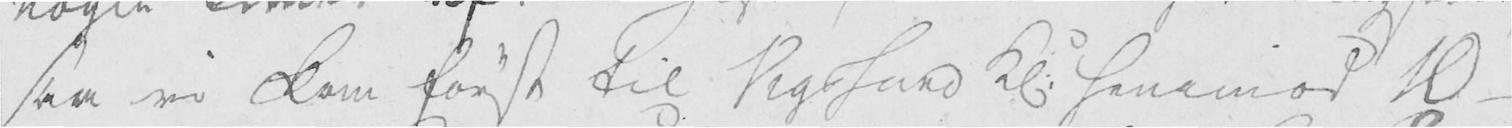saa vi kom først til Vigesund Kl. henimod 10 -
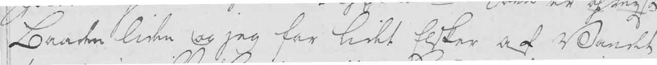Baaden liden og jeg for lidet Elsker af Vandet
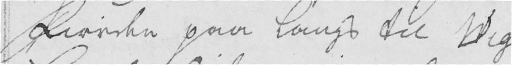Fiorden paa langs til Vig
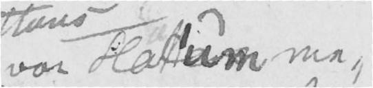van Hattum me-
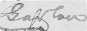Karlen
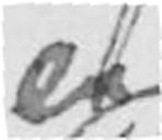er
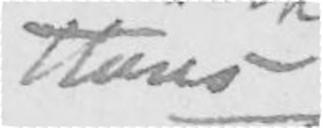Hans
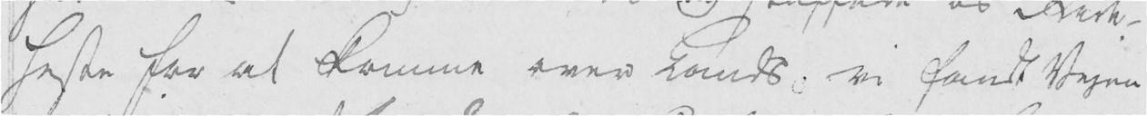heste for at komme over Lands; vi fandt Vejen
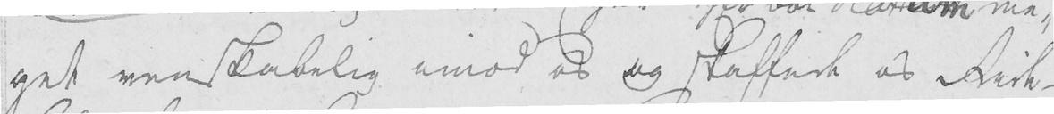get venskabelig imod os og skaffede os Ride-
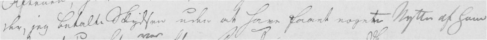der, jeg betalte Skydsen uden at have faaet noget Nytte af ham
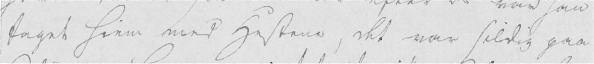taget hiem med Hestene, det var sildig paa
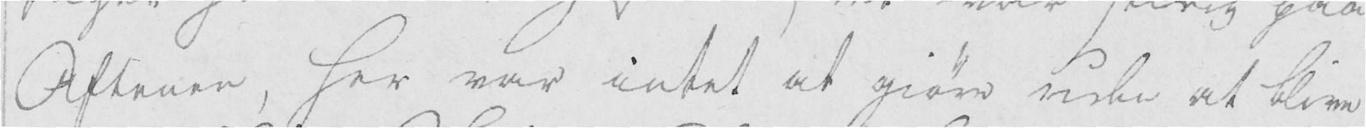Aftenen, her var intet at giøre uden at blive
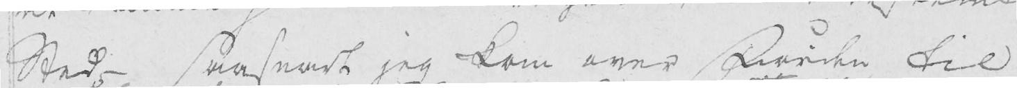Sted - Saasnart jeg kom over Fiorden til
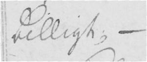billigt; -
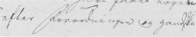efter Forordningen og gandske
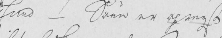sund - Søen er opreyst
